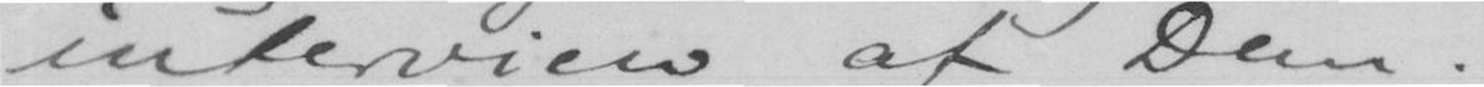interview af Dem.
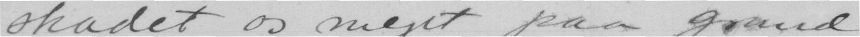skadet os meget paa grund
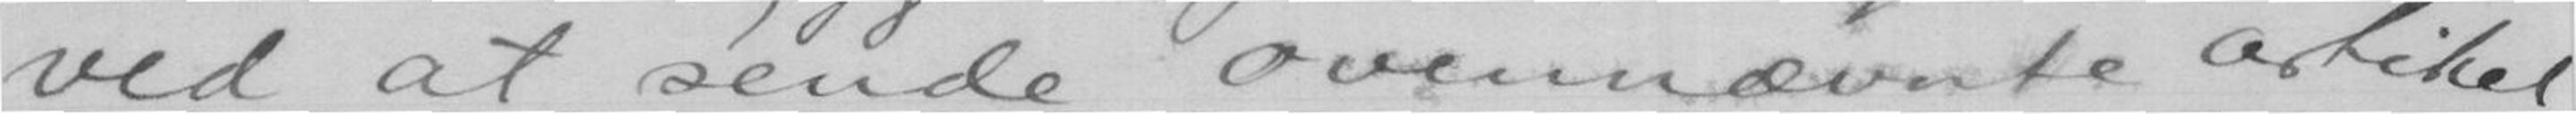ved at sende ovennævnte artikel
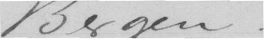Bergen.
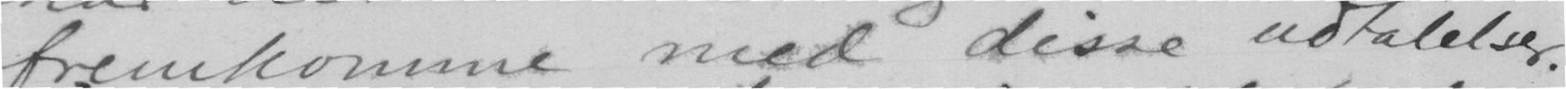fremkomme med disse udtalelser.
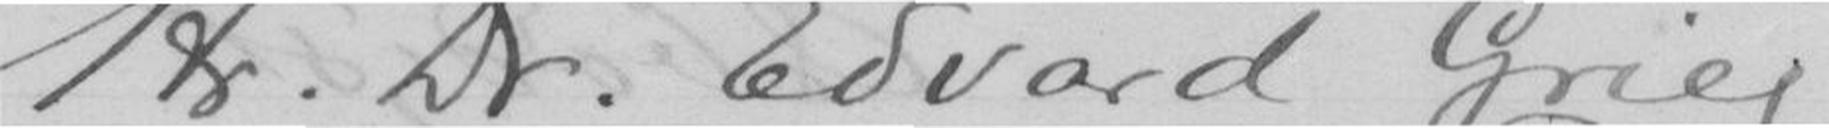Hr. Dr. Edvard Grieg,
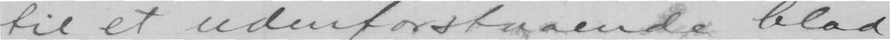til et udenforstaaende blad
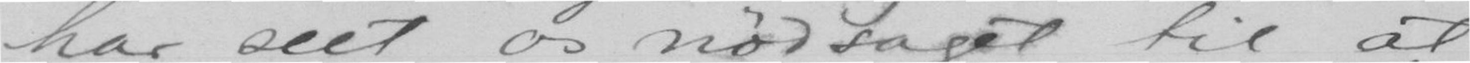har seet os nødsaget til at
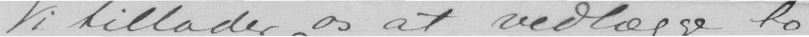Vi tillader os at vedlægge to
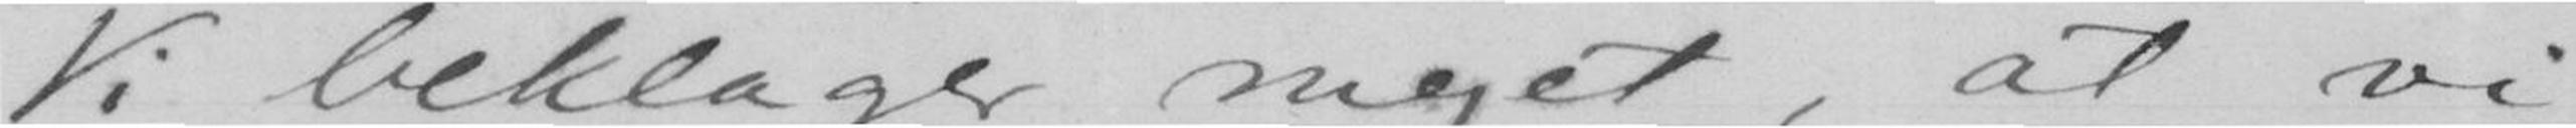Vi beklager meget, at vi
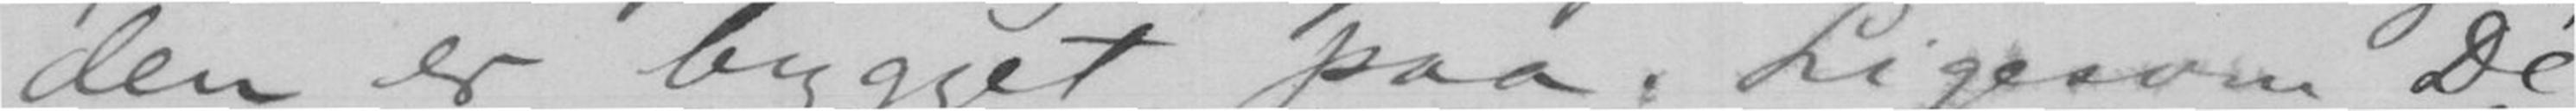den er bygget paa. Ligesom De
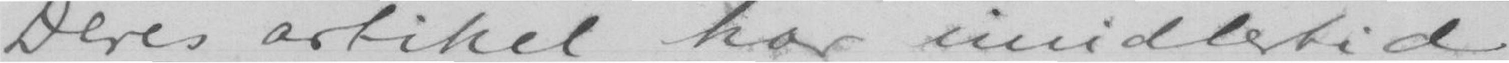Deres artikel har imidlertid
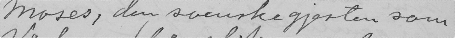Moses, den svenske gjesten som
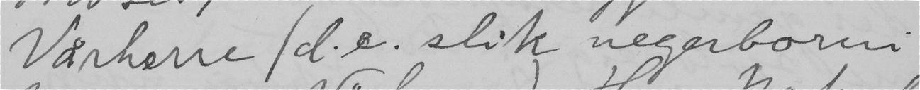Vårherre (d.e. slik negerborni
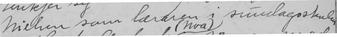Nielsen som læraren i sundagsskulen
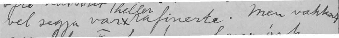vel segja var heller rafinerte. Men vakkert
